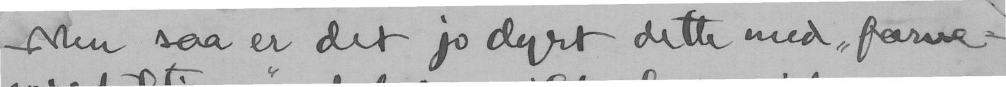Men saa er det jo dyrt dette med "farve-
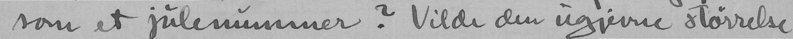som et julenummer? Vilde den ugjevne størrelse
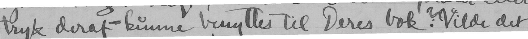tryk deraf - kunne benyttes til Deres bok? Vilde det
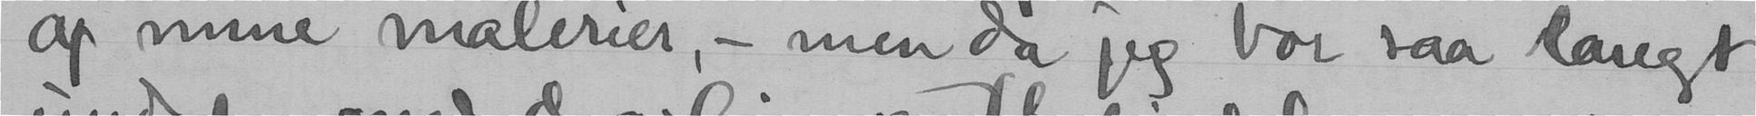af mine malerier, - men da jeg bor saa langt
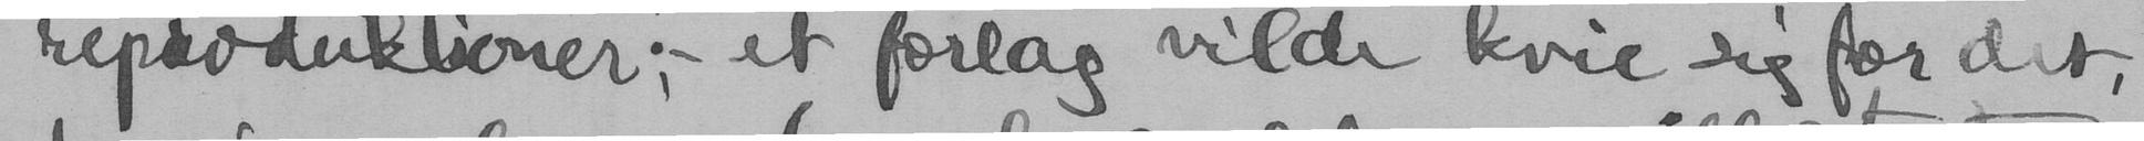repdoduktioner"; - et forlag vilde kvie sig for det,
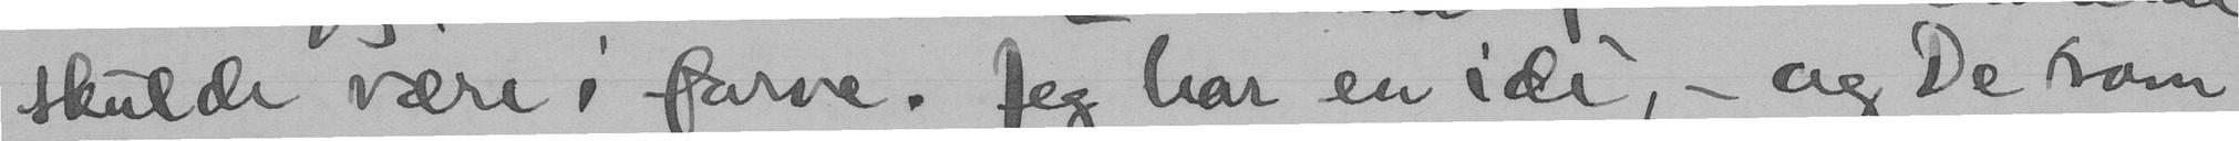skulde være i farve. Jeg har en ide, - og De som
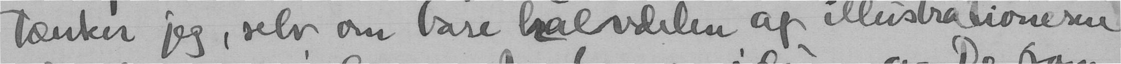tænker jeg, selv om bare halvdelen af illustrationerne
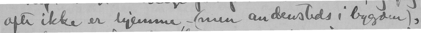ofte ikke er hjemme - (men andensteds i bygden),
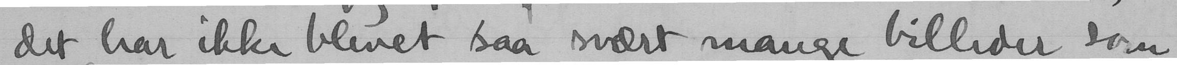det har ikke blevet saa svært mange billeder som
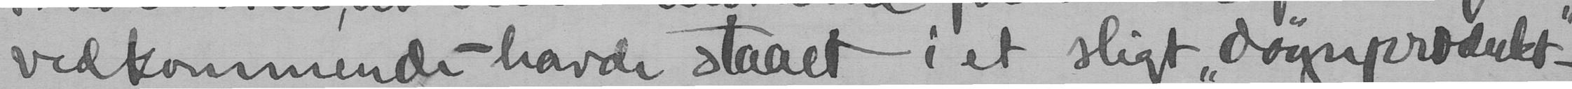vedkommende - havde staaet i et sligt "døgnprodukt",
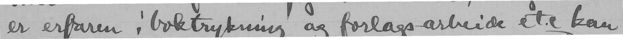er erfaren i boktrykning og forlagsarbeide etc. kan
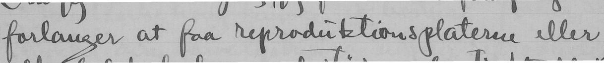forlanger at faa reproduktionsplaterne eller
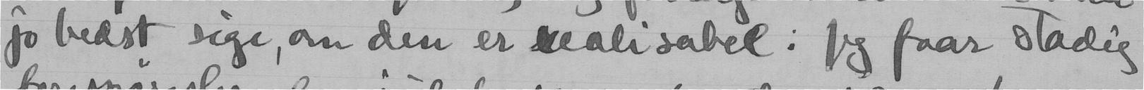jo bedst sige, om den er realesabel. Jeg faar stadig
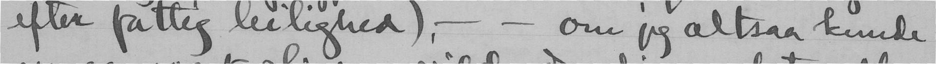efter fattig leilighed), - om jeg altsaa kunde
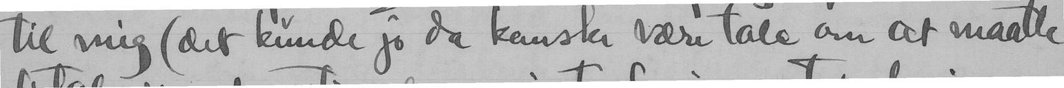til mig (det kunde jo da kanske være tale om at maatte
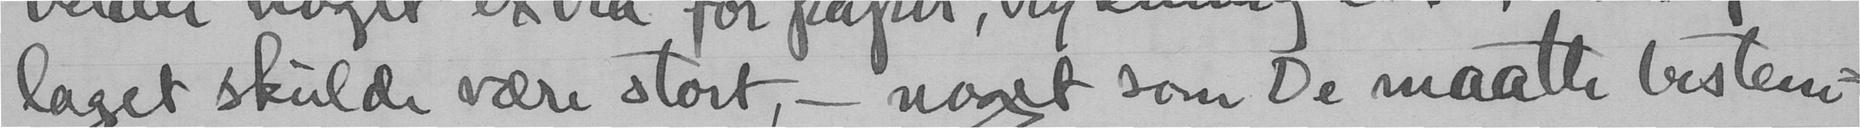laget skulde være stort, - noget som De maatte bestem-
